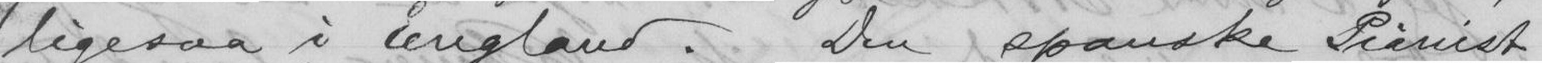ligesaa i England. Den spanske Pianist
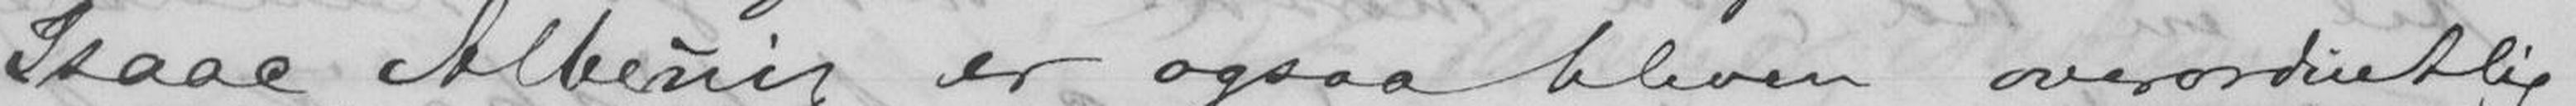Isaac Albenig er ogsaa bleven overordentlig
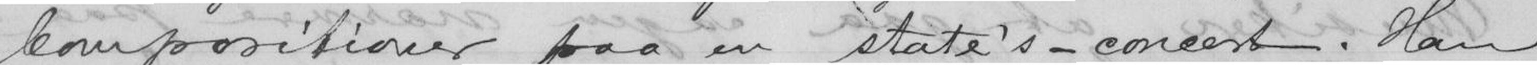Compositioner paa en state's-concert. Han
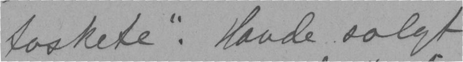toskete". Havde solgt
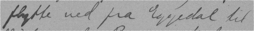flytte ned fra Eggedal til
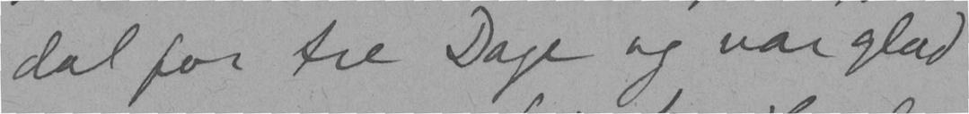dal for tre Dage og var glad
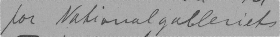for Nationalgalleriets
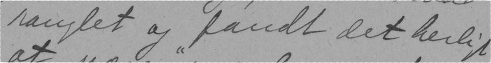ranglet og fandt det herligt
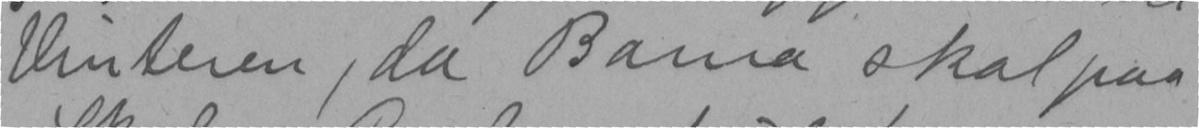Vinteren, da Barna skal paa
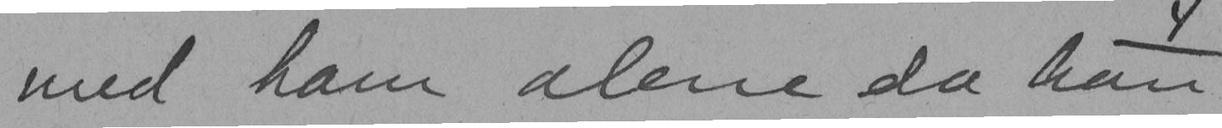med ham alene da han
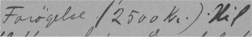Førøgelse (2500 Kr.) Vil
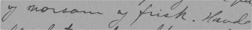og morsom og frisk. Havde
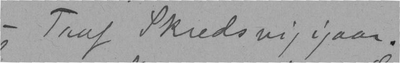- Traf Skredsvig igaar.
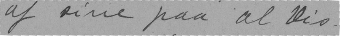af sine paa al Vis.
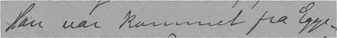Han var kommet fra Egge-
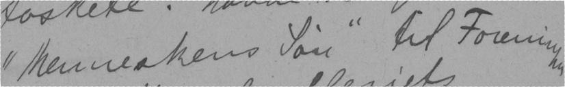"Menneskens Søn" til Foreningen
